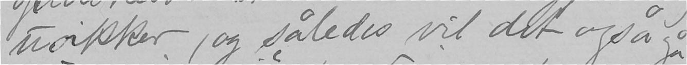usikker, og således vil det også gå
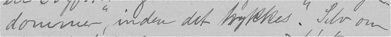dommer", inden det trykkes. Selv om
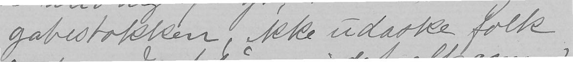gabestokken, ikke udæske folk,
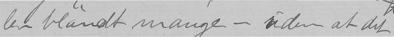ler blandt mange - uden at det
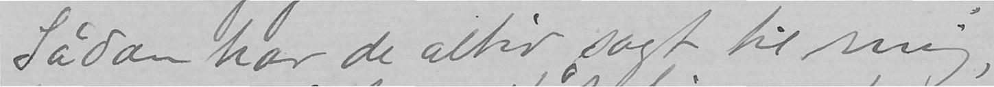Sådan har de altid sagt til mig,
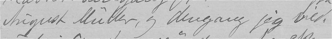August Müller, og dengang jeg vilde
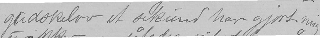gudskelov et sekund har gjort mig
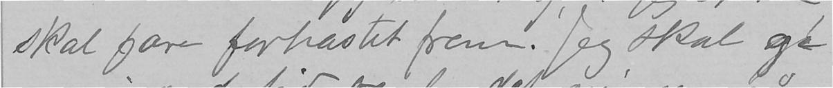skal fare forhastet frem. Jeg skal gi
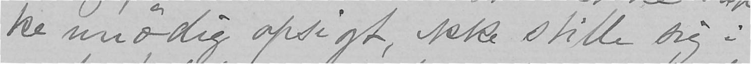ke unødig opsigt, ikke stille sig i
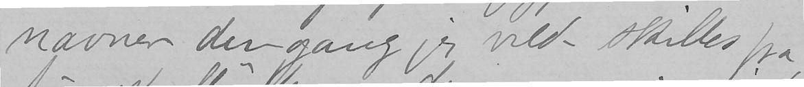nævner dengang jeg vilde skilles fra
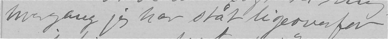hvergang jeg har ståt ligeoverfor
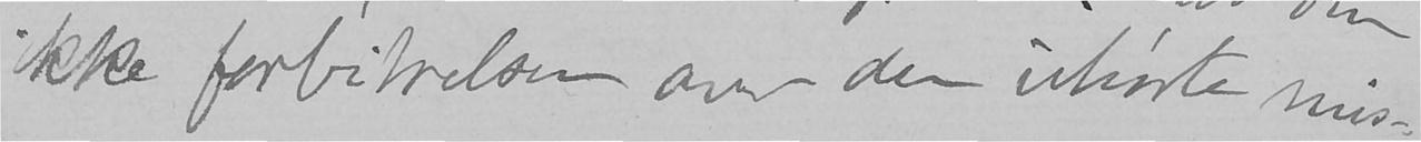ikke forbitrelsen over den uhørte mis-
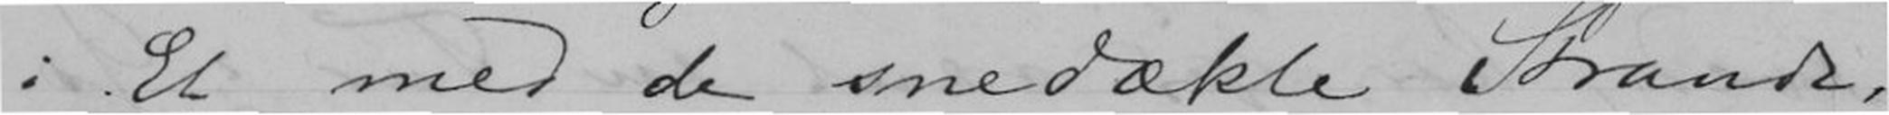i Et med de snedækte Strande,
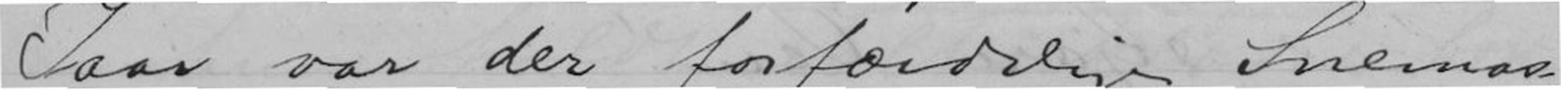Iaar var der forfærdelige Snemas-
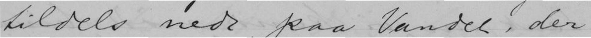tildels nede paa Vandet, der
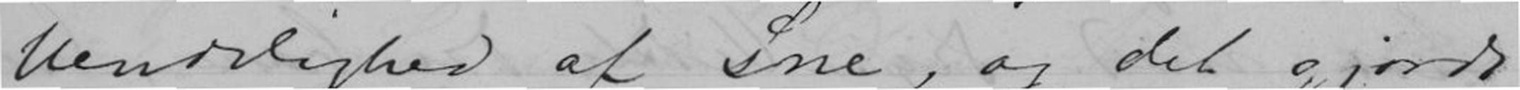Uendelihed af Sne, og det gjorde
